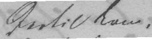Dertil kom,
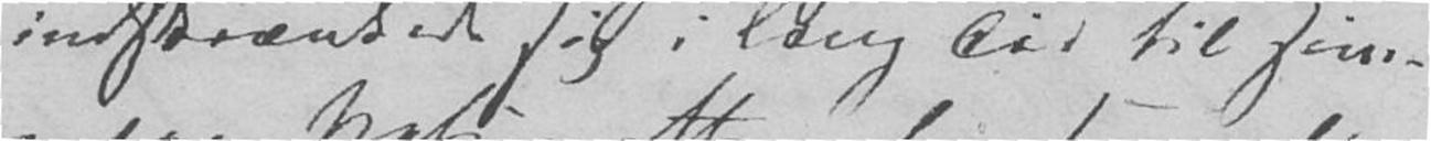indskrænkede sig i lang Tid til sim-
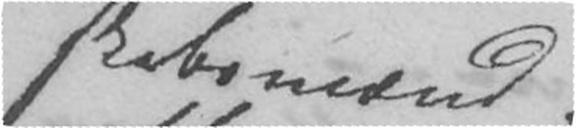skabsmænd
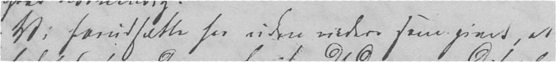Vi forudsætte her uden videre som givet, at
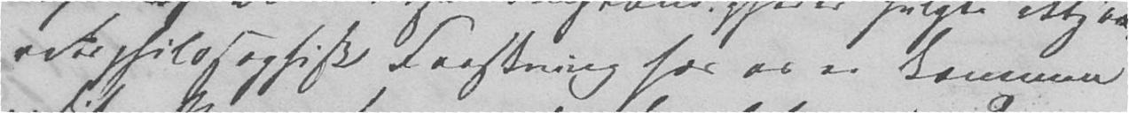retsphilosophisk Forskning hos os er kommen
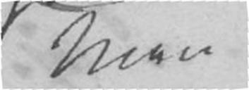Men
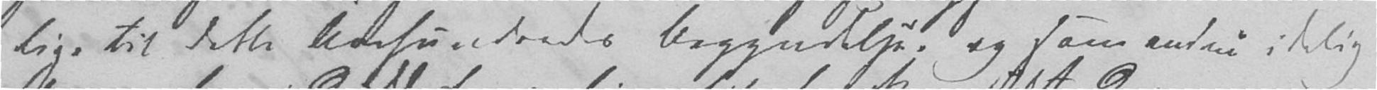lige til dette Aarhundredes Begyndelse, og som endnu idelig
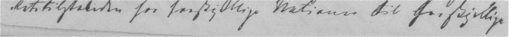Retstilstanden hos forskjellige Nationer til forskjellige
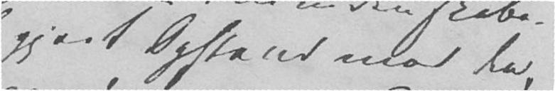gjort Opstand mod den,
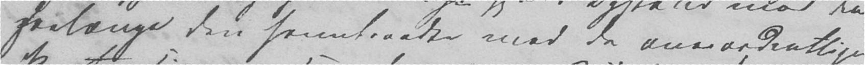saalænge den fremtraadte med de overordentlige
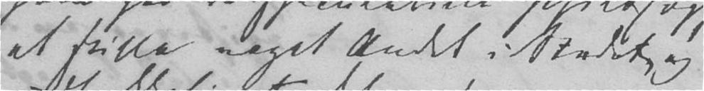at stille noget Andet i Stedet, og
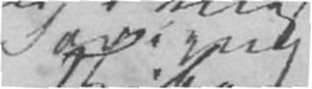Savigny
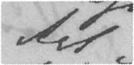Ret
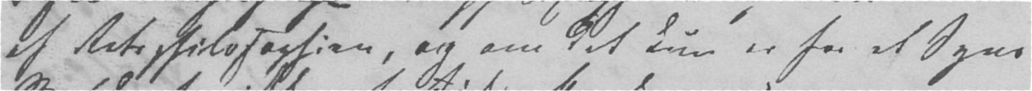af Retsphilosophien, og om det kun er for et Syns
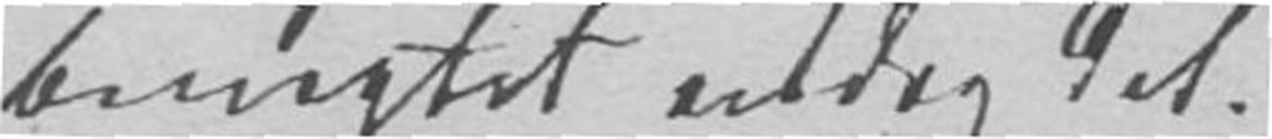benægtet endog det.
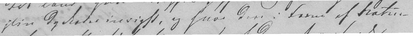plin dyrkedes ivrigst, og hvor den i Form af Natur-
